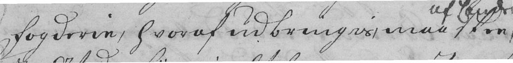Fogderie, hvoraf udbringis, maa skee
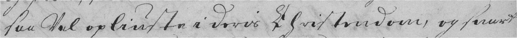saa vel opliuste i deris Christendom, og snart
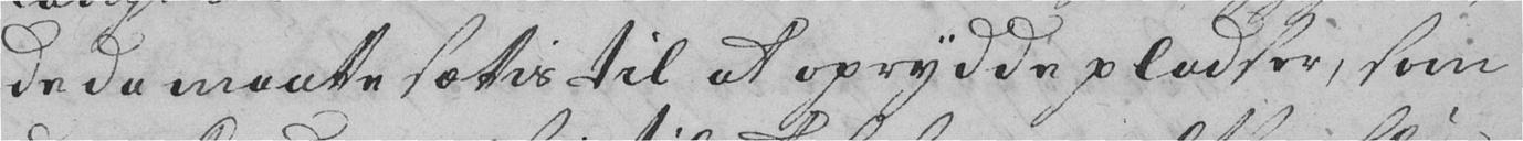de da maatte sættes til at oprydde pladser, som
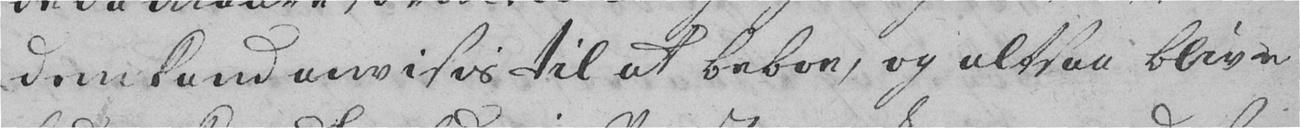den kand anvisis til at beboe, og altsaa blive
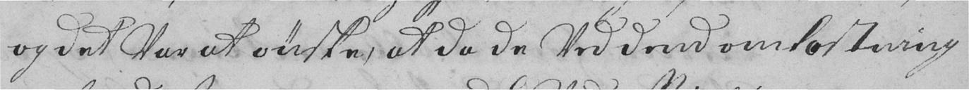og det var at ønske, at da de Ved dend omkostning
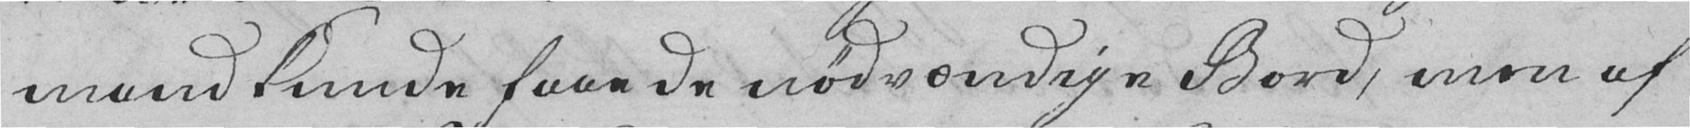mand kunde faae de nødvændige Bord, men af
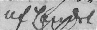af Landet
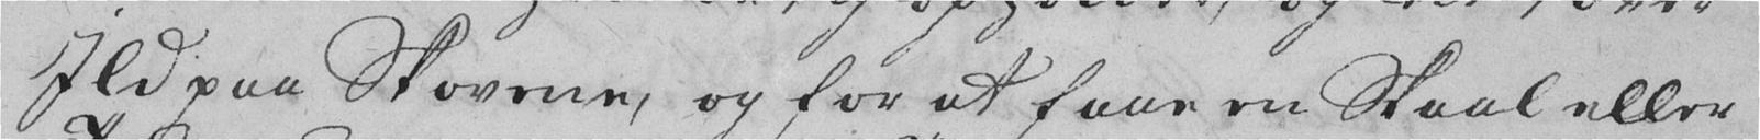Ild paa Skovene, og for at faae en Skaal eller
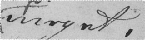meget.
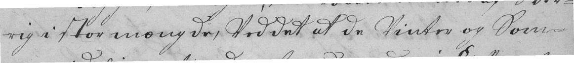rig i stor mængde, ved det at de Vinter og Som-
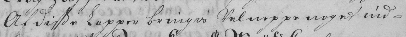Af disse Lapper bringis Vel neppe noget ind-
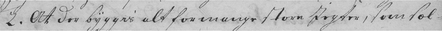2. At der byggis alt for mange store Iegter, som sæl-
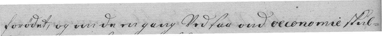forødet, og om de en gang Ved saa ond oeconomie skul-
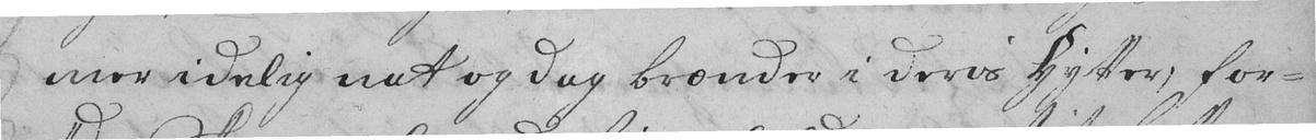mer idelig nat og dag brænder i deris Hytter, for-
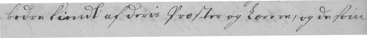bedre kiendt af deris Præster og Lærere, og de som
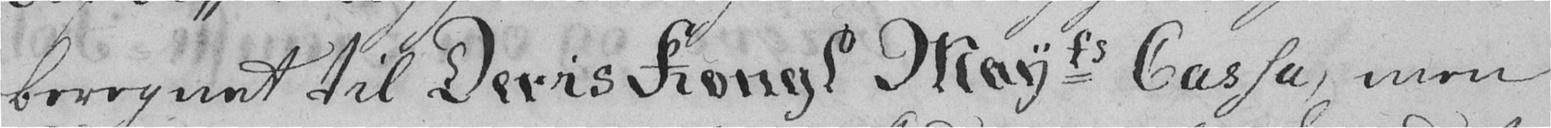beregnet til Deris Kongl Mayts Cassa, men
